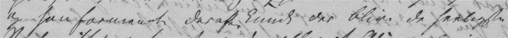og han formeente derefter kunde der blive de herligste
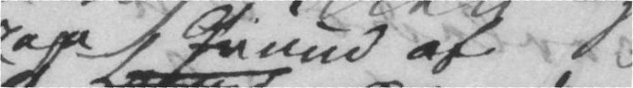paa Grund af
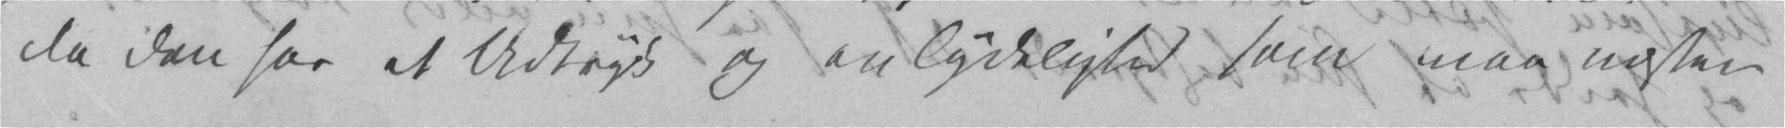da den har et Udtryk og en Tydelighed som man næsten
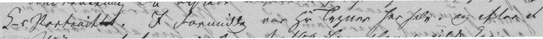C-s Portraittet. I Formiddag var Hr Tegner her selv. og efter et
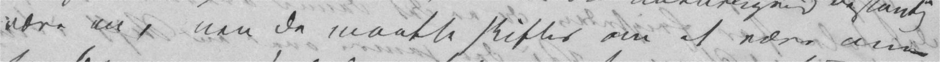være en, men de maatte skiftes om at være om
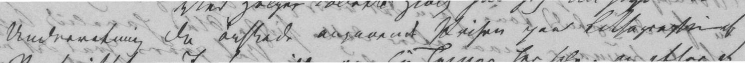Underretning Du ønskede angaaende Prisen paa Lithographie af
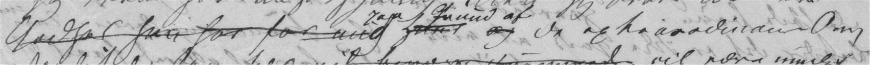Godhed hun har for mig Huus og de extraordinaire Om
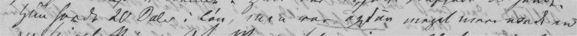Hun havde 20 Daler i Løn, men var ogsaa meget mere værdt end
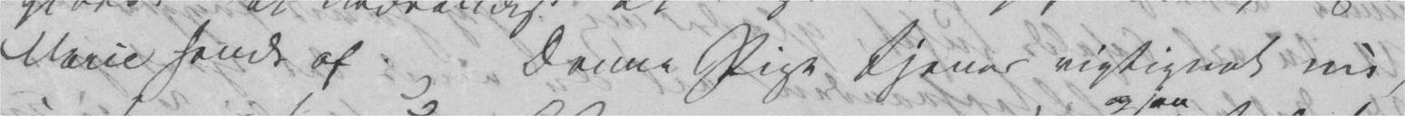Marie hende af. Denne Pige tjener rigtignok nu,
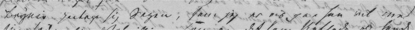Brynie paatage sig Sagen, som jeg er vis paa han vil med
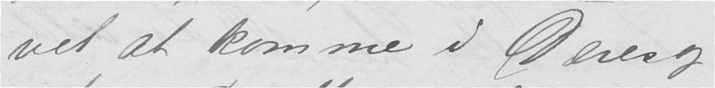vel at komme i Deres og
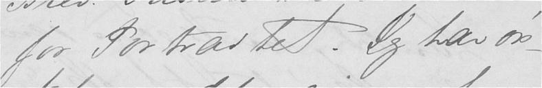for Portrætet. Jeg har øn-
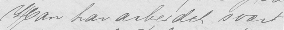Han har arbeidet svært
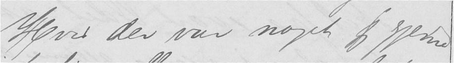Hvis der var noget jeg gjerne
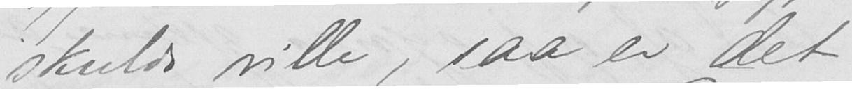skulde ville, saa er det
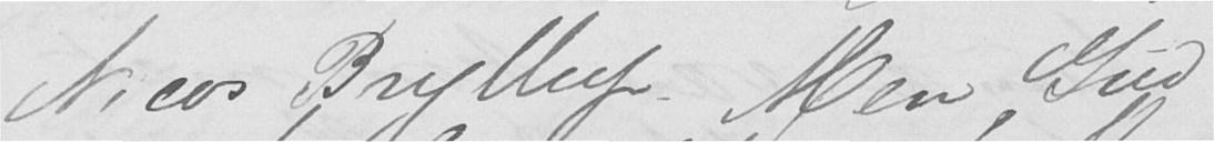Nicos Bryllup. Men Gud
